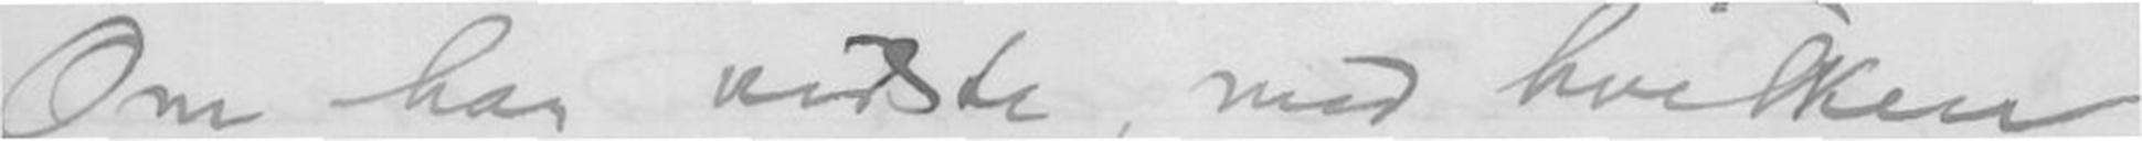Om han vidste, med hvilken
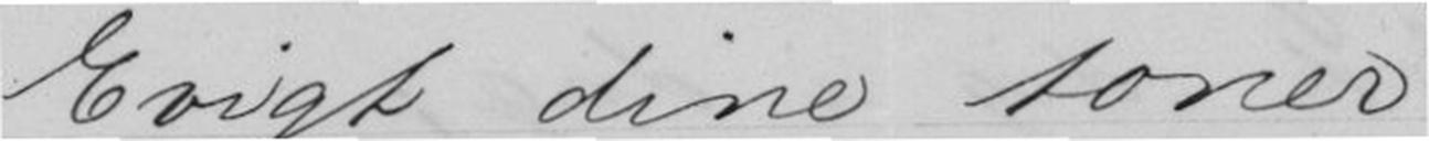Evigt dine toner
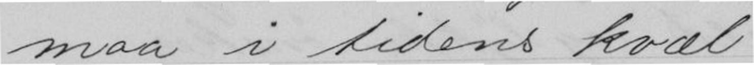maa i tidens kval
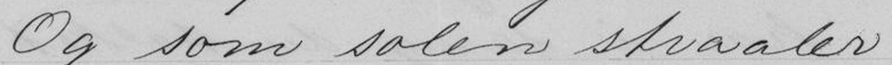Og som solen straaler
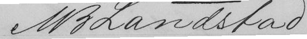MB Landstad
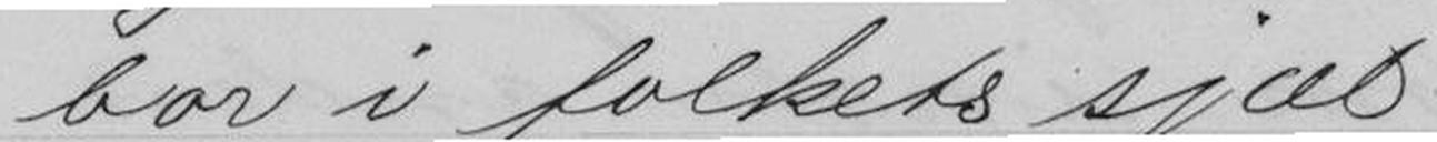bor i folkets sjæl
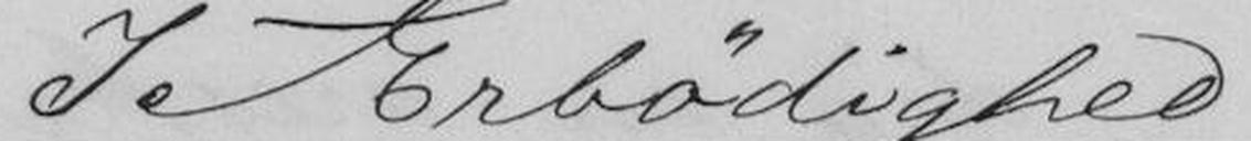I Ærbødighed
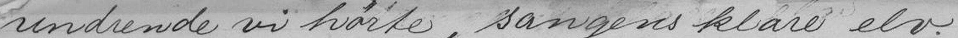undrende vi hørte sangens klare elv
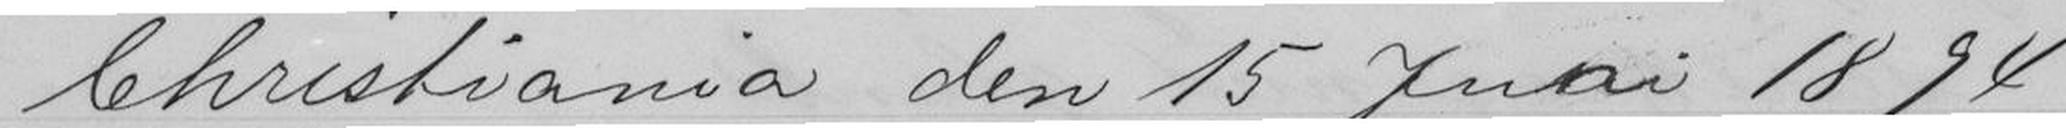Christiania den 15 juni 1894
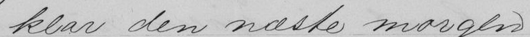klar den næste morgen
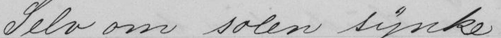Selv om solen synke
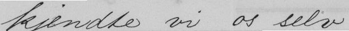kjendte vi os selv
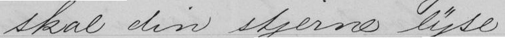skal din stjerne lyse
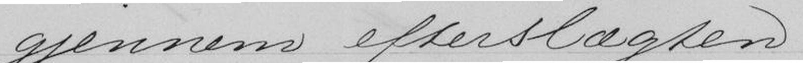gjennem efterslægten
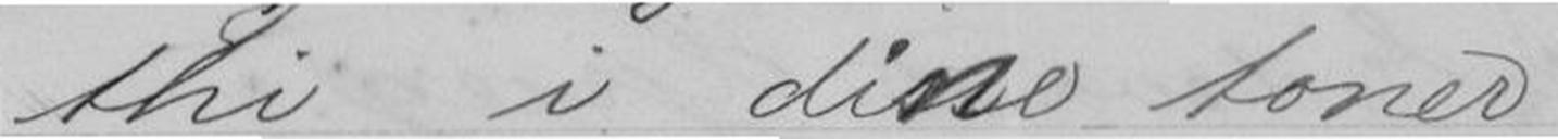thi i disse toner
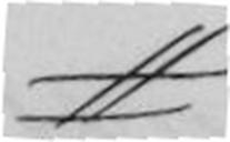#
